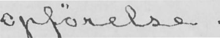opførelse.
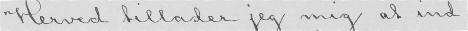Herved tillader jeg mig at ind-
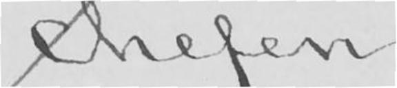Chefen
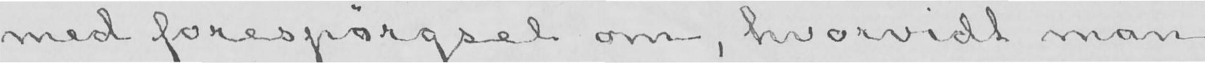med forespørgsel om, hvorvidt man
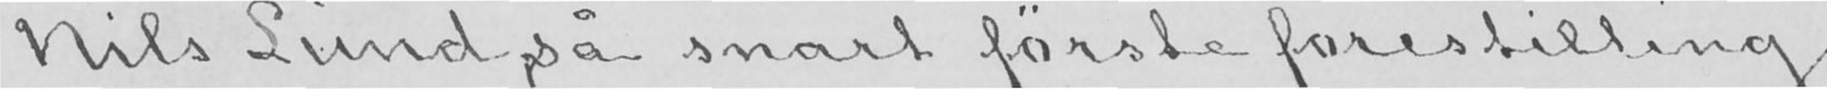Nils Lund ,så snart første forestilling
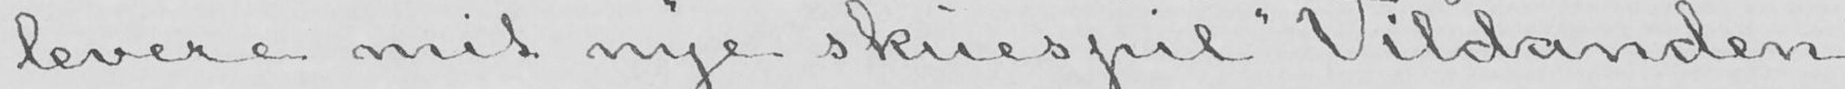levere mit nye skuespil «Vildanden»
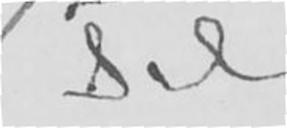Til
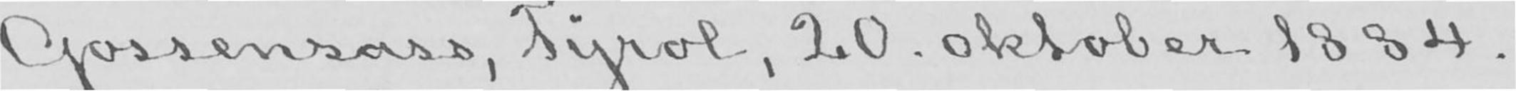Gossensass, Tyrol, 20. oktober 1884.
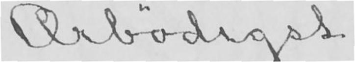Ærbødigst
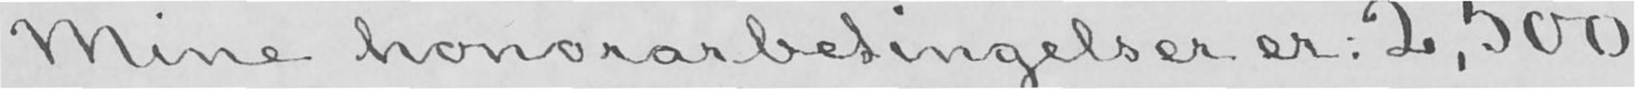Mine honorarbetingelser er: 2,500
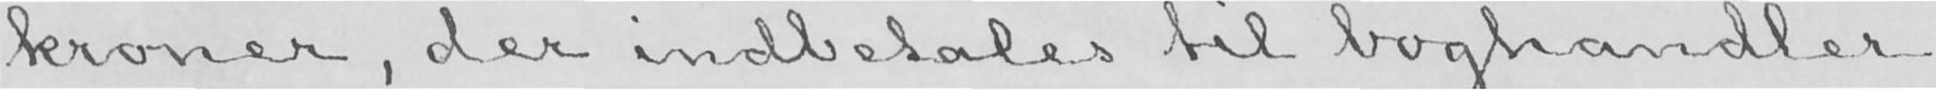kroner, der indbetales til boghandler
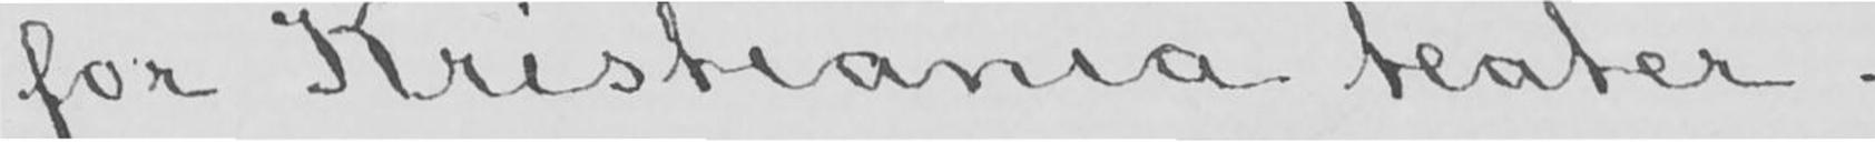for Kristiania teater.
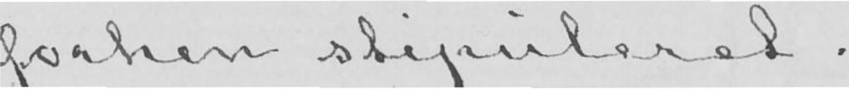forhen stipuleret.
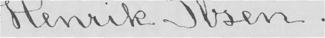Henrik Ibsen.
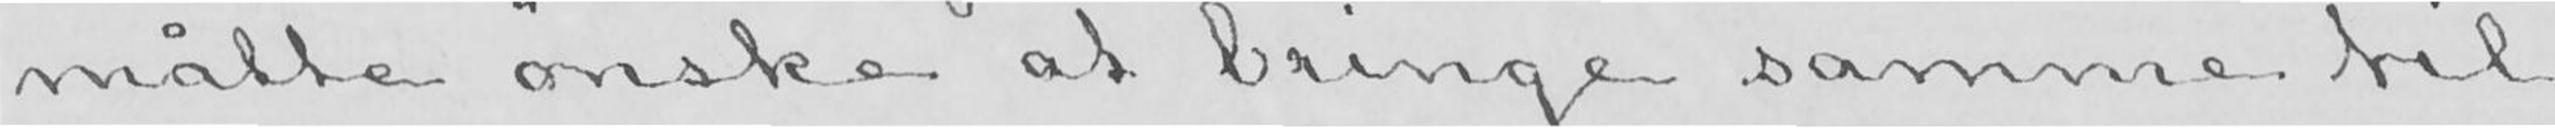måtte ønske at bringe samme til
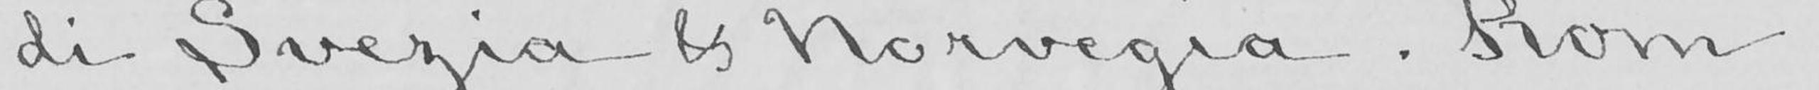di Svezia & Norvegia, Rom.
